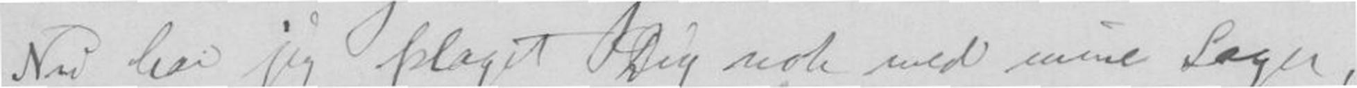Nu har jeg plaget Dig nok med mine Sager,
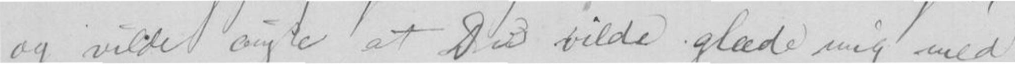og vilde ønske at Du vilde glæde mig med
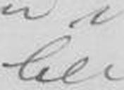her
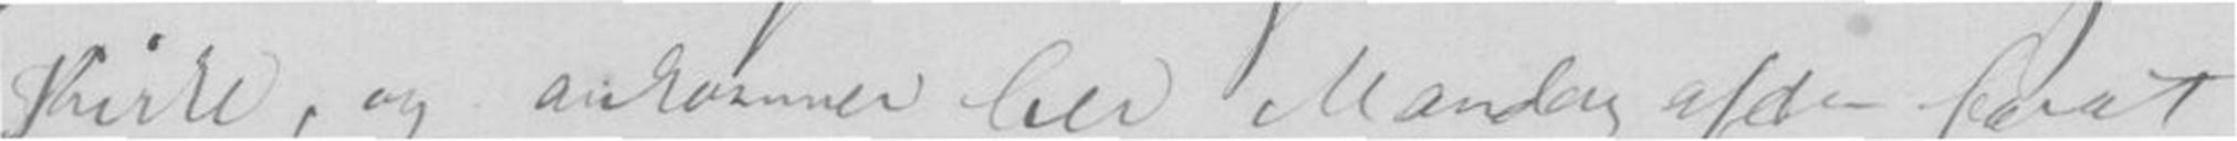Kirke, og ankommer her Mandag aften forat
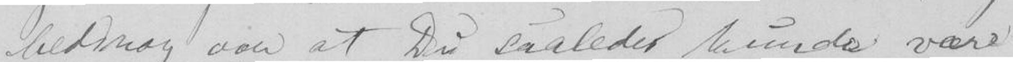hetsnag von at Du saaledes kunde være
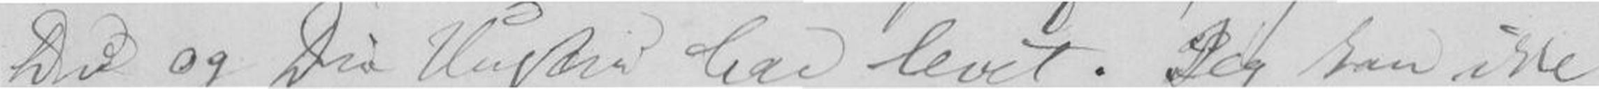Du og Din Hustru har levet. Jeg kan ikke
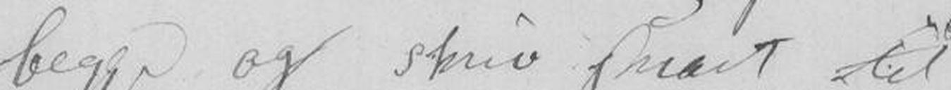begge og skriv snart til
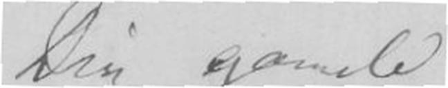Din gamle
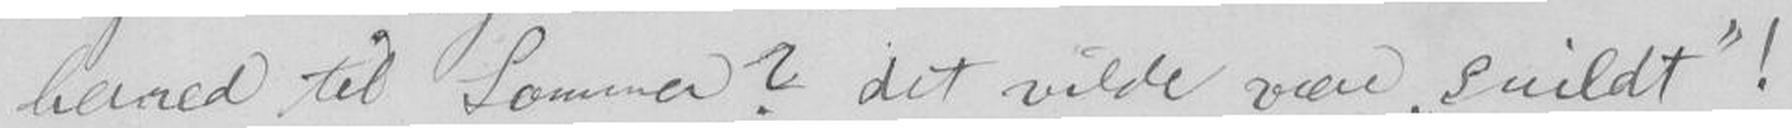herned til Sommeren? det vilde være snildt!
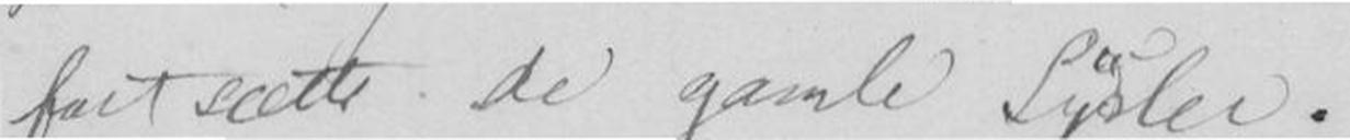fortsætte de gamle Sysler.
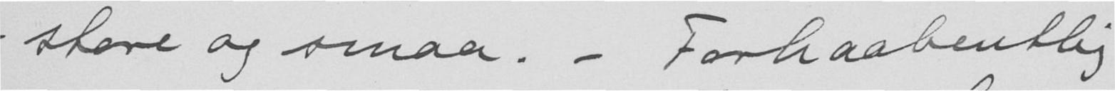- store og smaa. - Forhaabentlig
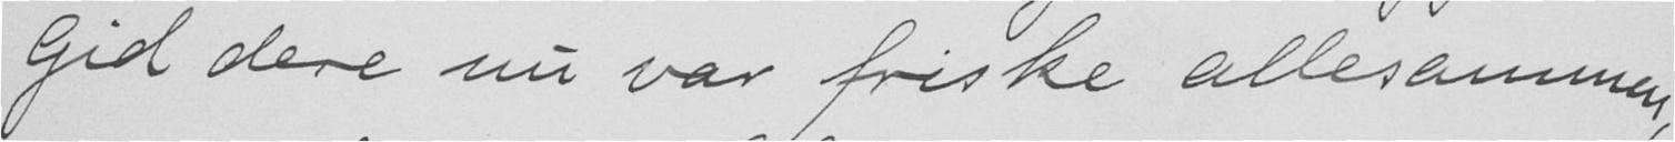Gid dere nu var friske allesammen,
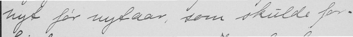nyt før nytaar, som skulde for-
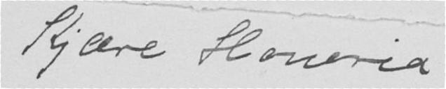Kjære Honoria!
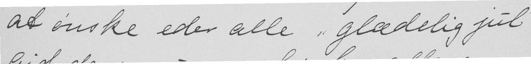at ønske eder alle "glædelig jul"
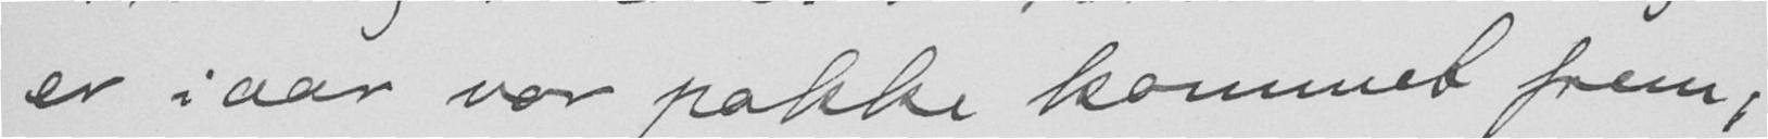er i aar vor pakke kommet frem,
